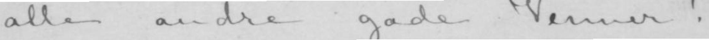alle andre gode Venner!
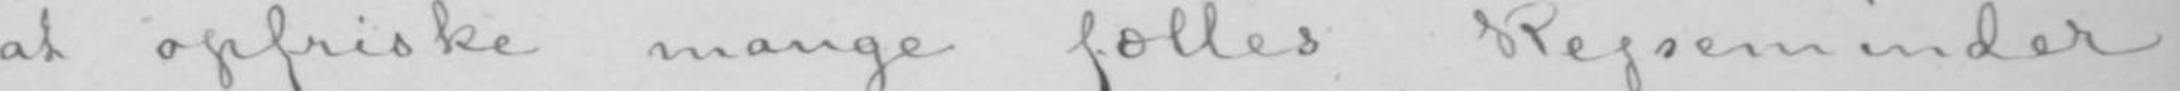at opfriske mange fælles Rejseminder,
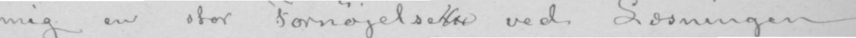mig en stor Fornøjelsen ved Læsningen
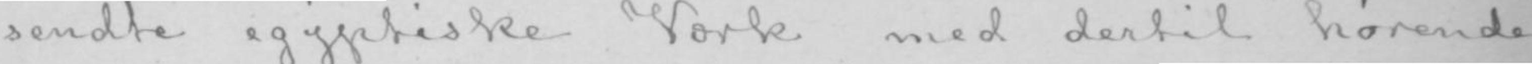sendte egyptiske Værk med dertil hørende
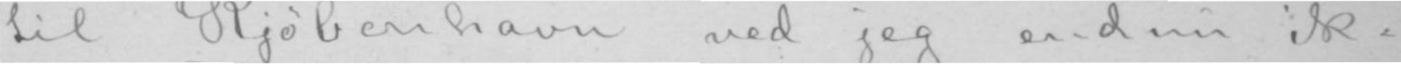til Kjøbenhavn ved jeg endnu ik-
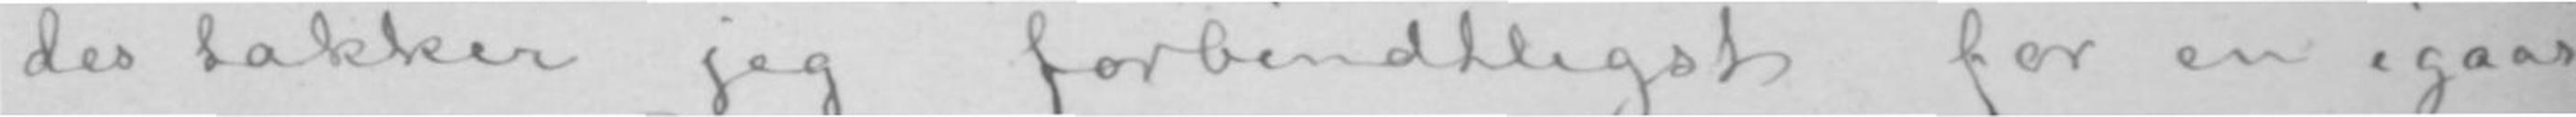des takker jeg forbindtligst for en igaar
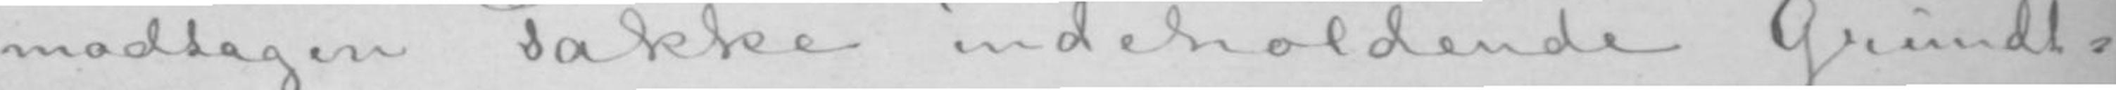modtagen Pakke indeholdende Grundt-
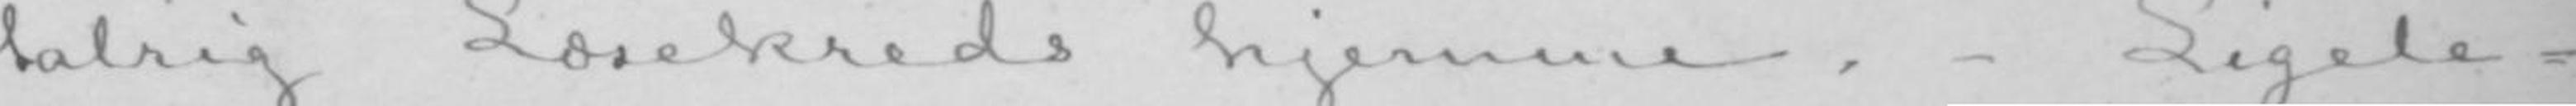talrig Læsekreds hjemme.- Ligele-
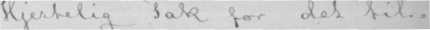Hjertelig Tak for det til-
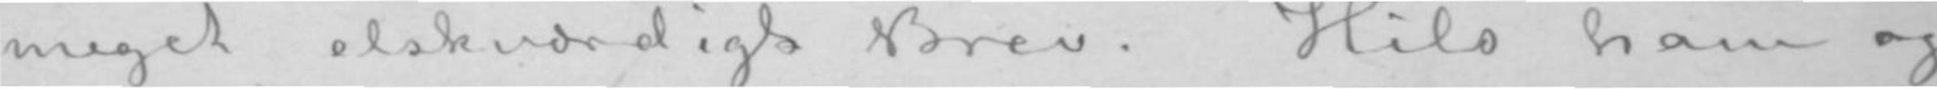meget elskværdigt Brev. Hils ham og
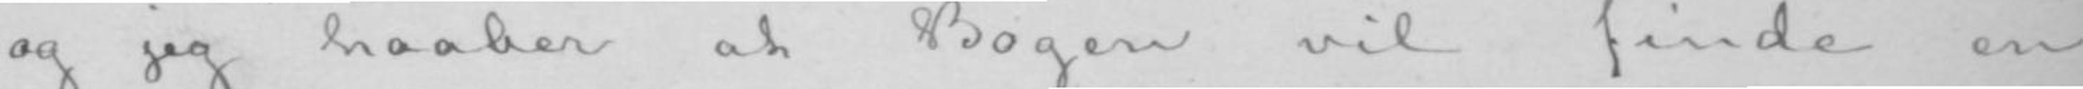og jeg haaber at Bogen vil finde en
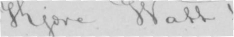Kjære Watt!
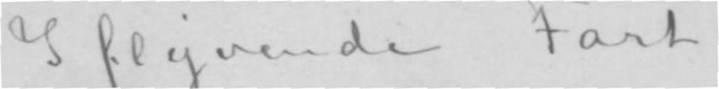I flyvende Fart
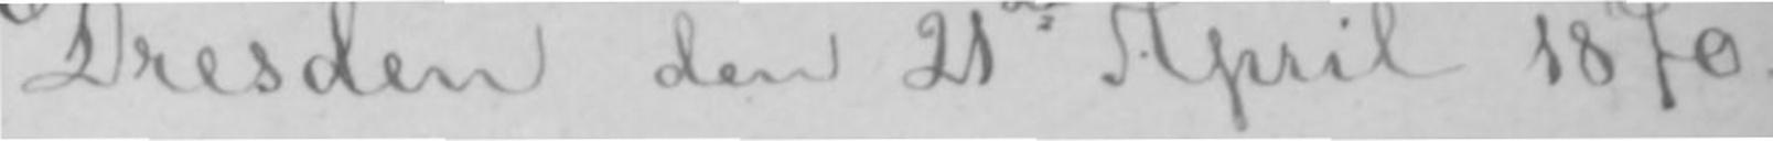Dresden den 21de April 1870.-
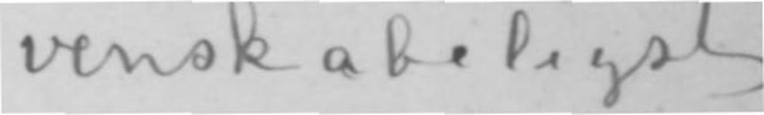venskabeligst
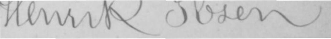Henrik Ibsen.
